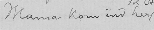Mama kom ind her
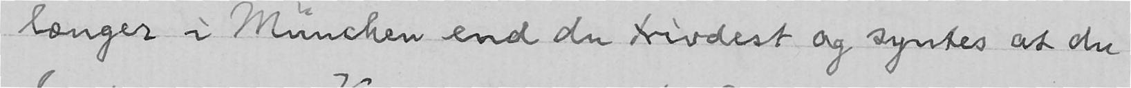længer i München end du trivdest og syntes at du
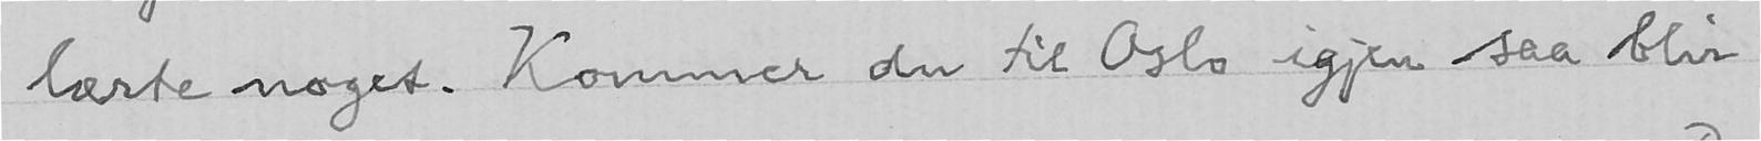lærte noget. Kommer du til Oslo igjen saa blir
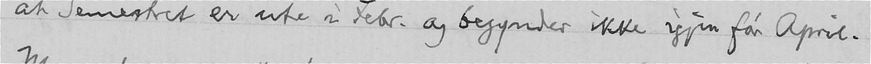at Semestret er ute i Febr. og begynder ikke igjen før April.
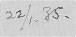22/1. 35.
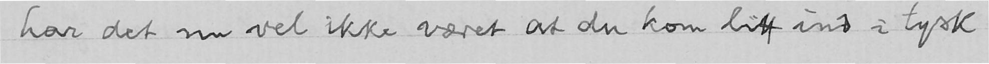har det nu vel ikke været at du kom litt ind i tysk
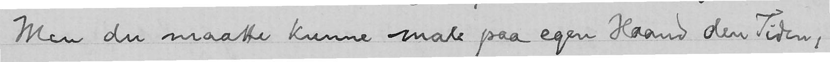Men du maatte kunne male paa egen Haand den Tiden,
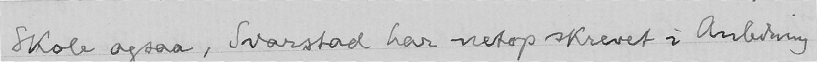Skole ogsaa, Svarstad har netop skrevet i Anledning
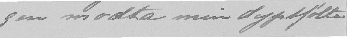gen modta min dyptfølte
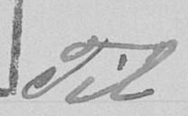Til
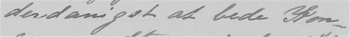derdanigst at bede Kon-
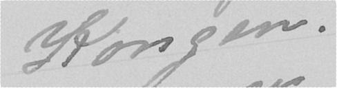Kongen.
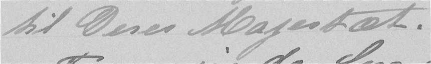til Deres Majestæt.
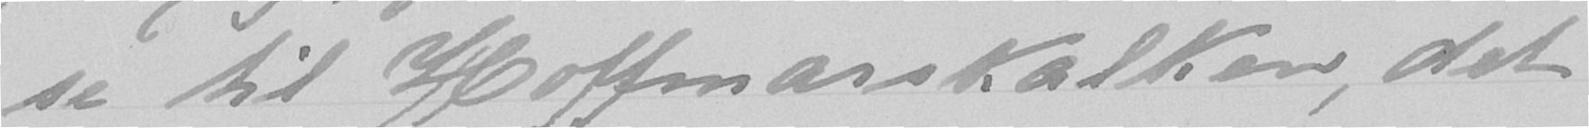se til Hoffmarskalken, det
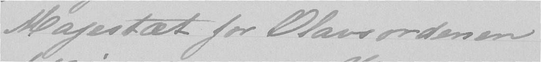Majestæt for Olavsordenen
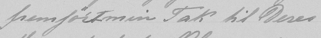frem først min Tak til Deres
